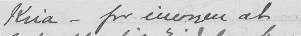Kria - for imorgen at
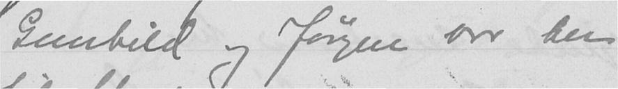Gunhild og Jørgen var her
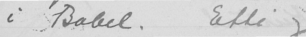i Babel. Etti og
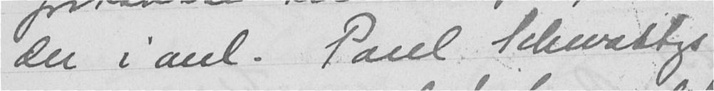der i anl. Paul Schwartzs
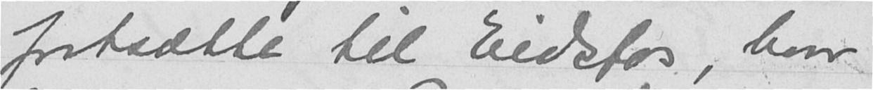fortsætte til Eidsfos, hvor
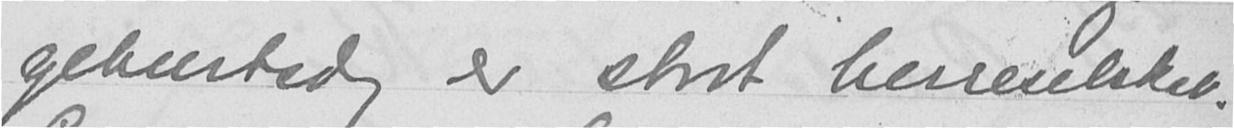geburtsdag er stort herreselskab.
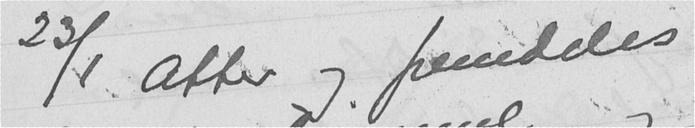23/1 Atter og fremdeles
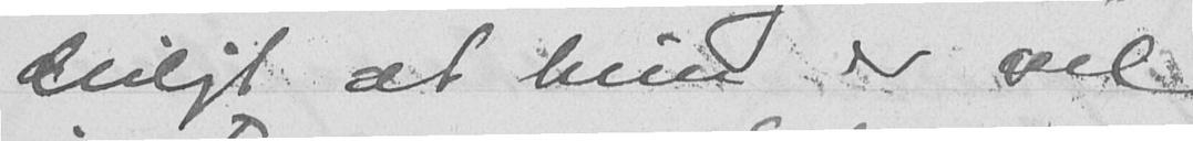deiligt at hun er vel
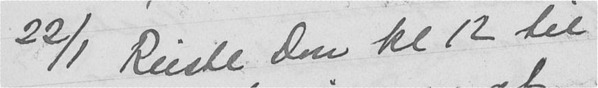22/1 Reiste Don kl 12 til
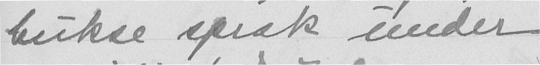bukse sprak under
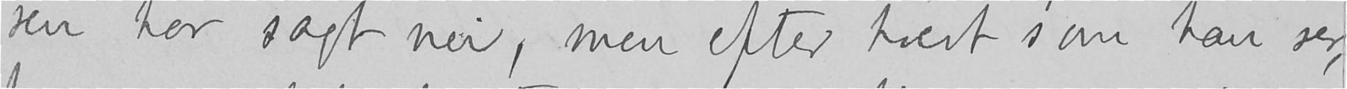ren har sagt nei, men efter hvert som han ser,
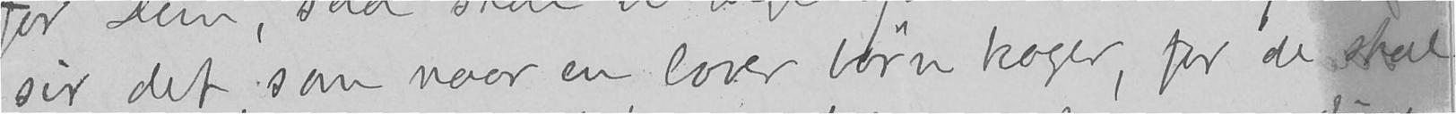sir det, som naar en lover børn kager, for de skal
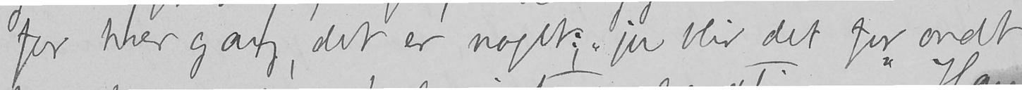for hver gang, det er noget: "ja blir det for ondt
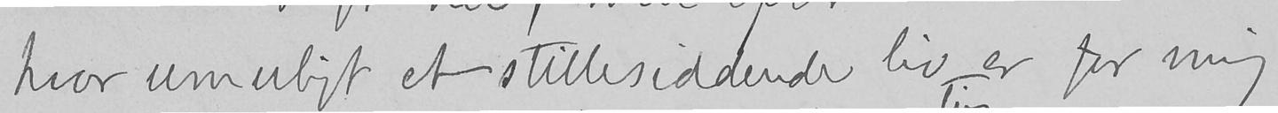hvor umuligt et stillesiddende liv er for mig,
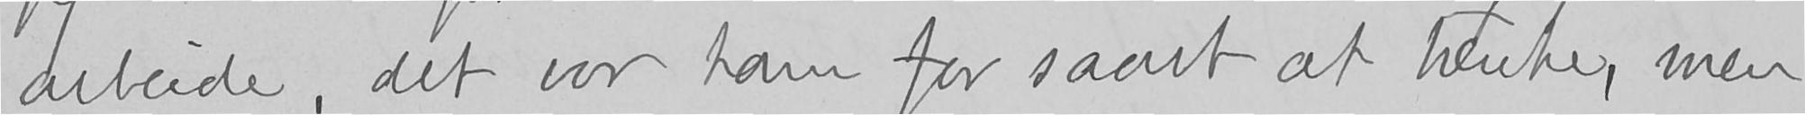arbeide, det var ham for saart at tænke, men
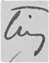ting
